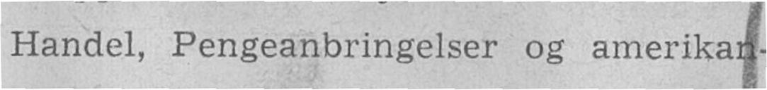Handel, Pengeanbringelser og amerikan-
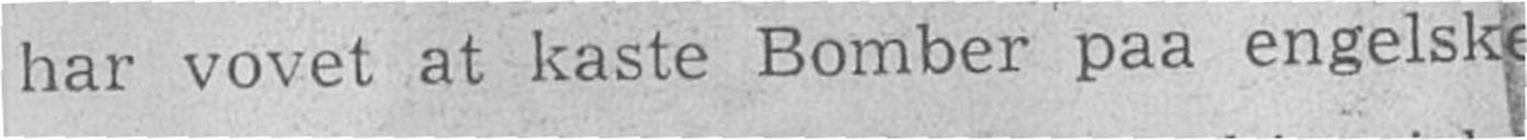har vovet at kaste Bomber paa engelske
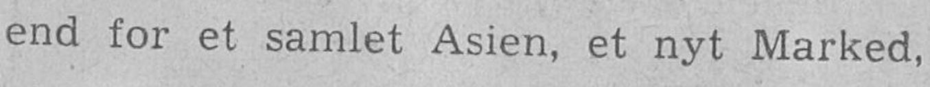end for et samlet Asien, et nyt Marked,
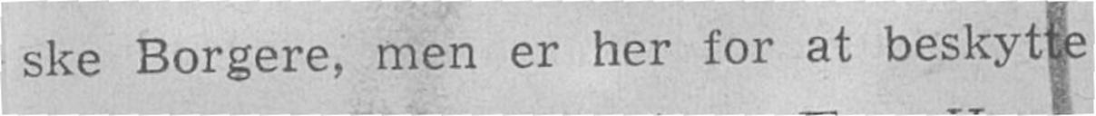ske Borgere, men er her for at beskytte
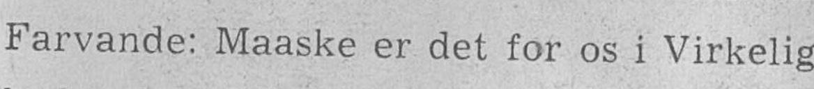Farvande: Maaske er det for os i Virkelig-
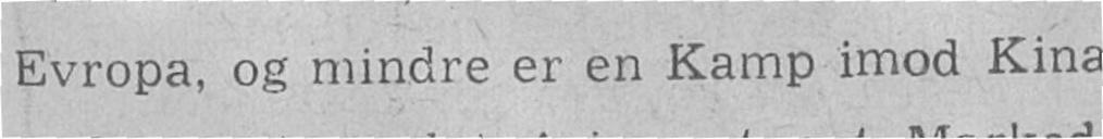Evropa, og mindre er en Kamp imod Kina
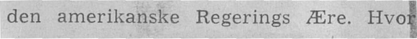den amerikanske Regerings Ære. Hvor-
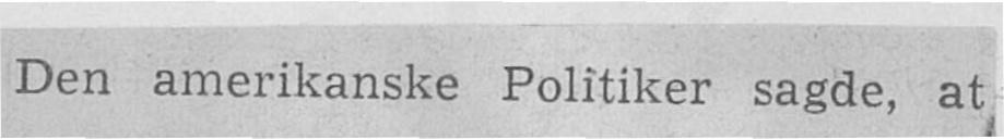Den amerikanske Politiker sagde, at
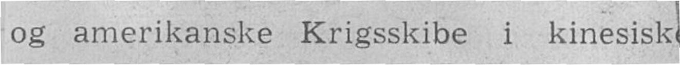og amerikanske Krigsskibe i kinesiske
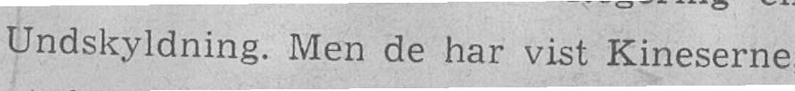Undskyldning. Men de har vist Kineserne,
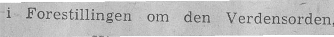i Forestillingen om den Verdensorden,
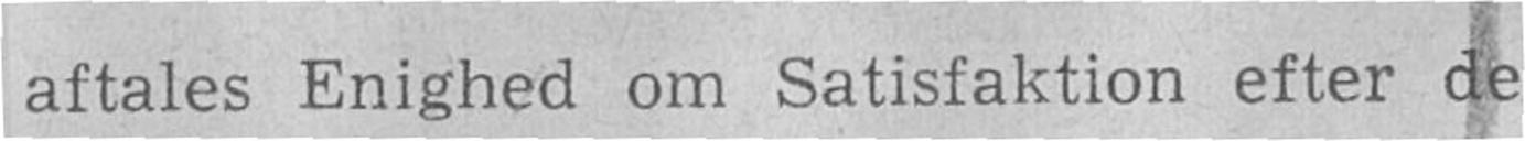aftales Enighed om Satisfaktion efter de
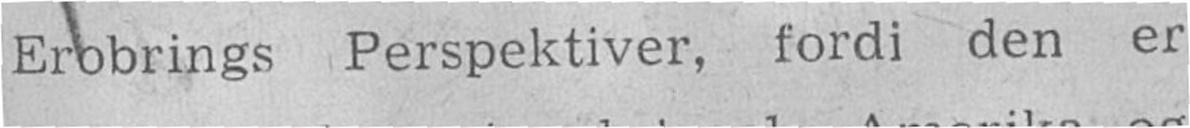Erobrings Perspektiver, fordi den er
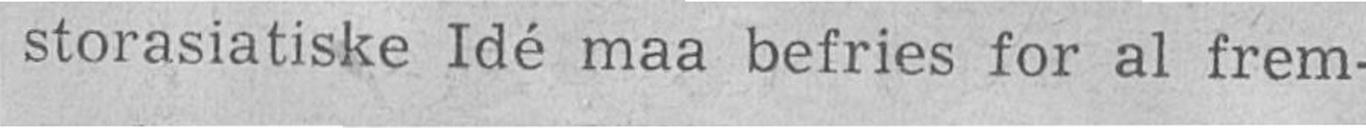storasiatiske Idé maa befries for al frem-
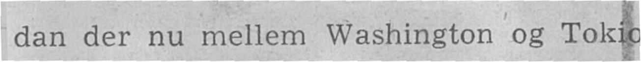dan der nu mellem Washington og Tokio
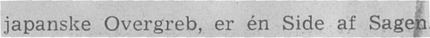japanske Overgreb, er én Side af Sagen.
